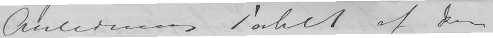Anledning Tabet af din
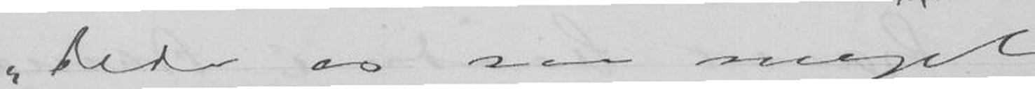dede os saa meget
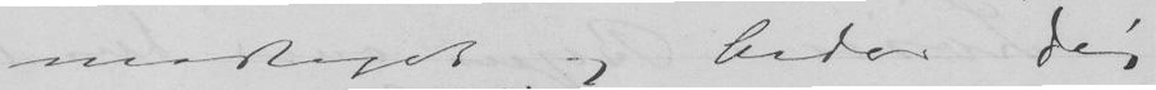modtaget og beder Dig
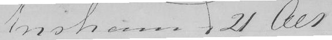Kristiania d 21 Oct
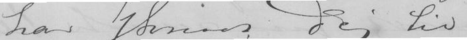har skrevet Dig til
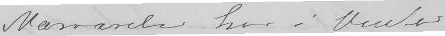Nærværelse her i Vinter
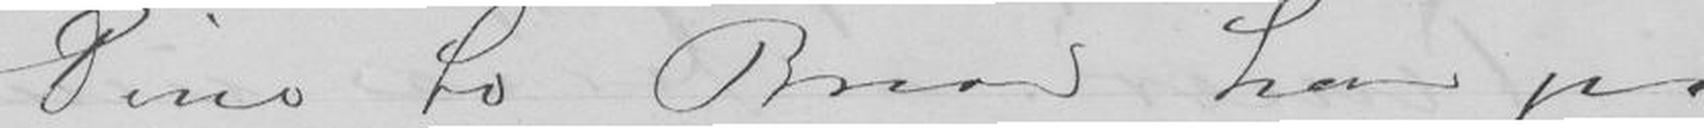Dine to Brev har jeg
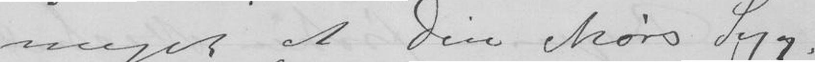meget at Din Mors Syg-
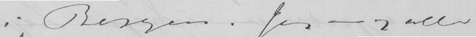i Bergen. Jeg - og alle
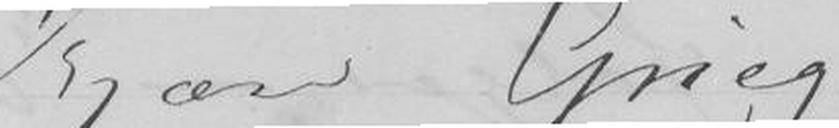Kjære Grieg
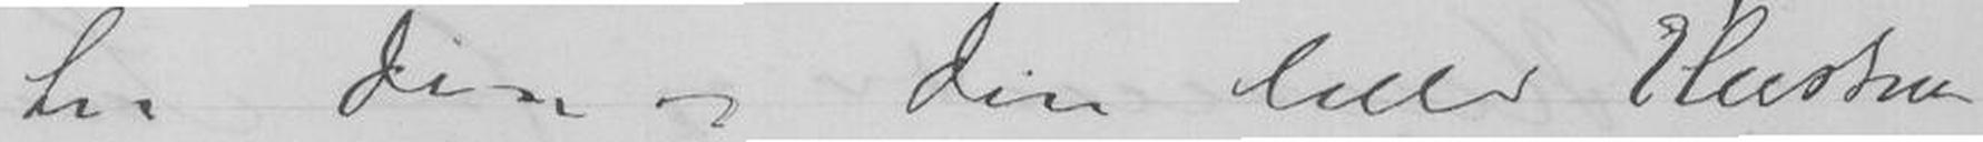til Din og Din lille Hustrus
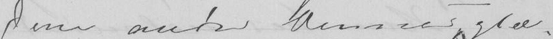Dine andre Venner glæ-
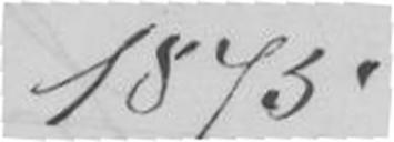1875
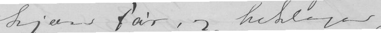kjære Far, og beklager
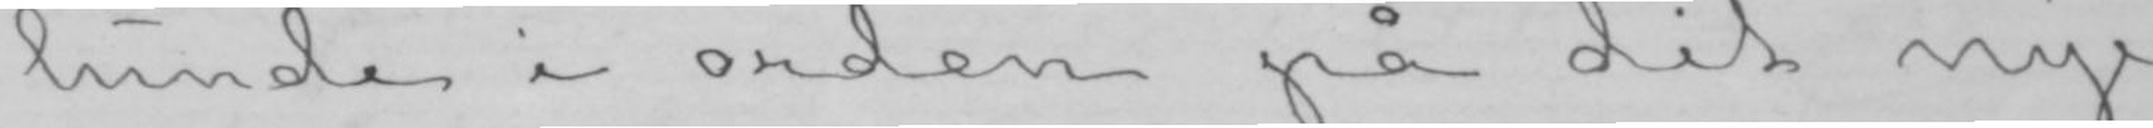lunde i orden på dit nye
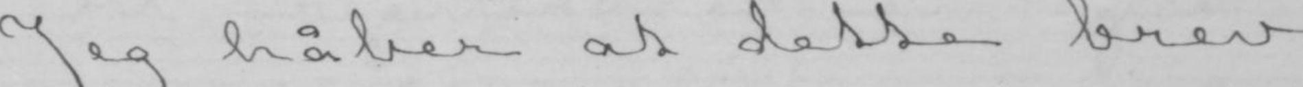Jeg håber at dette brev
